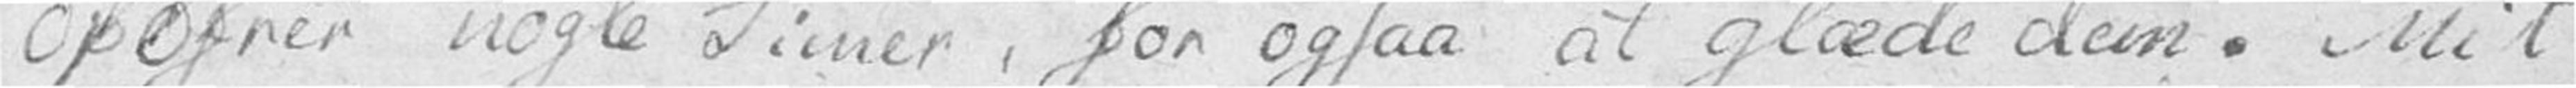opofrer nogle Timer, for ogsaa at glæde dem. Mit
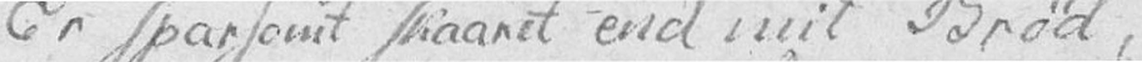Er sparsomt skaaret end mit Brød,
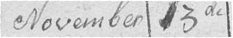November 13de
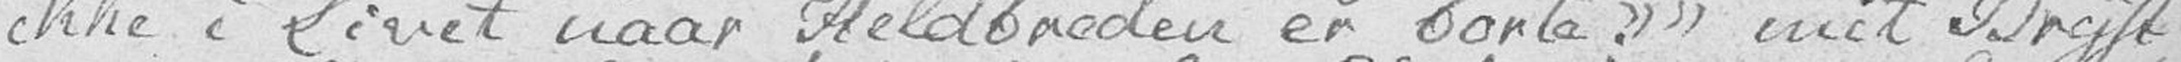ikke i Livet naar Heldbreden er borte."" mit Bryst
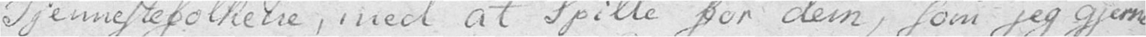Tjennestefolkene, med at Spille for dem, som jeg gjerne
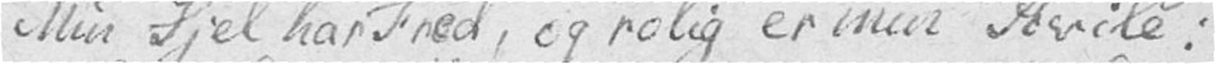Min Sjel har Fred, og rolig er min Hvile:
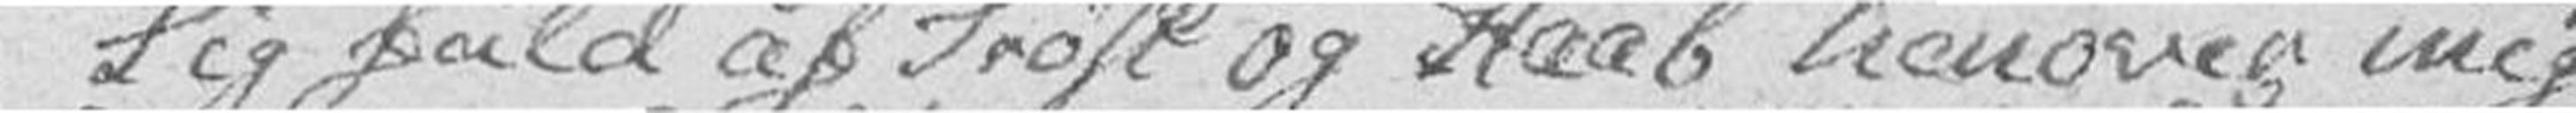Sig fuld af Trøst og Haab henover mig
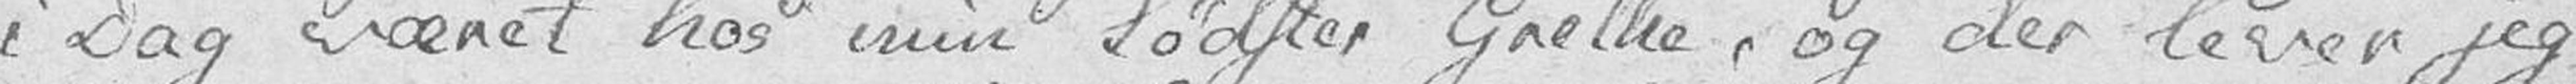i Dag været hos min Sødster Grethe, og der lever jeg
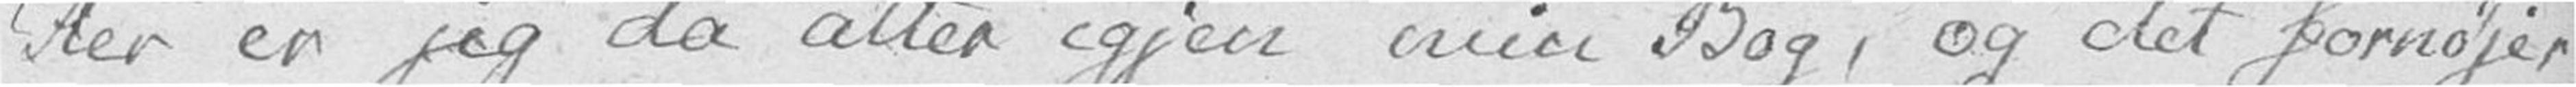Her er jeg da atter igjen min Bog, og det fornøjer
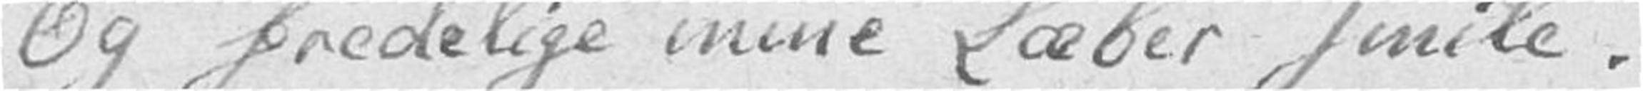Og fredelige mine Læber smile.
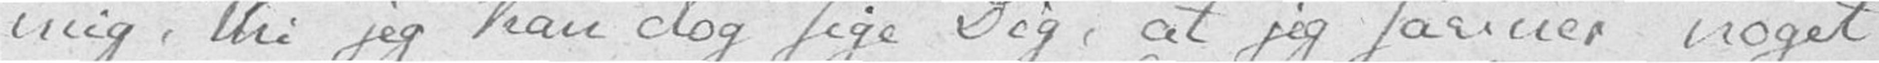mig, thi jeg kan dog sige Dig, at jeg savner noget
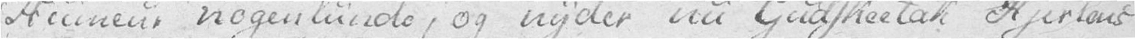Humeur nogenlunde, og nyder nu Gudskeetak Hjertens
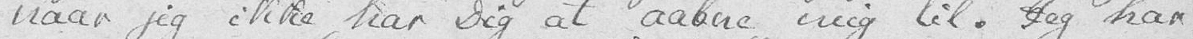naar jeg ikke har Dig at aabne mig til. Jeg har
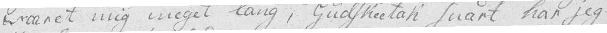været mig meget lang, Gudskeetak snart har jeg
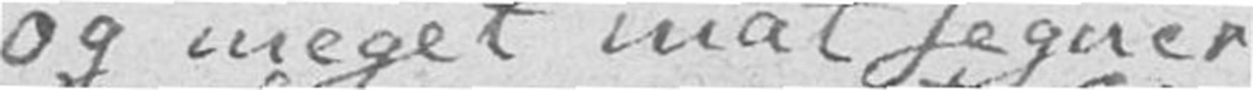og meget mat segner
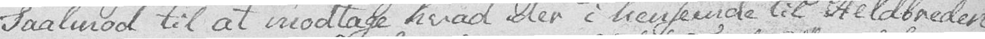Taalmod til at modtage hvad der i henseende til Heldbreden
